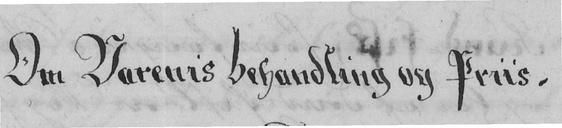Om Varenis behandling og Priis.
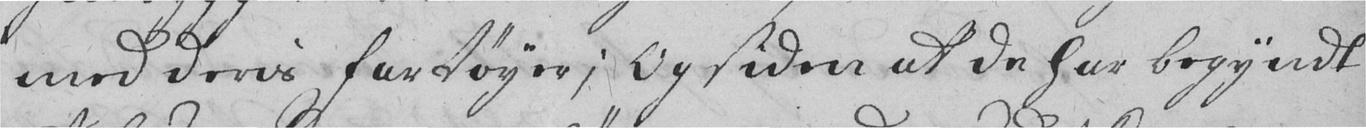med deris Fartøyer; Og siden at de har begyndt
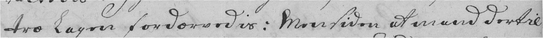træ Lagen fordærvedis: Men siden at mand dertil
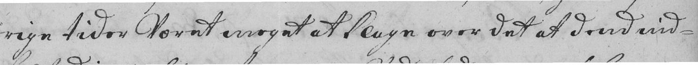rige tider Været meget at klage over det at dend ind-
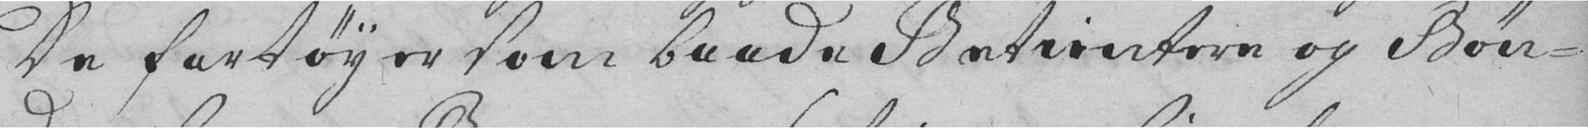De fartøyer som baade Betientere og Bøn-
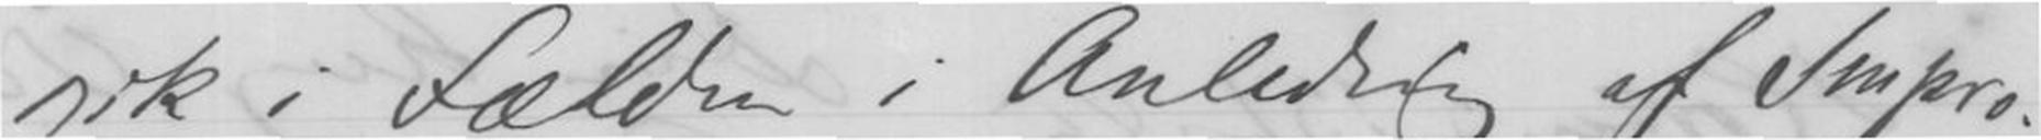gik i Fælden i Anledning af Impro
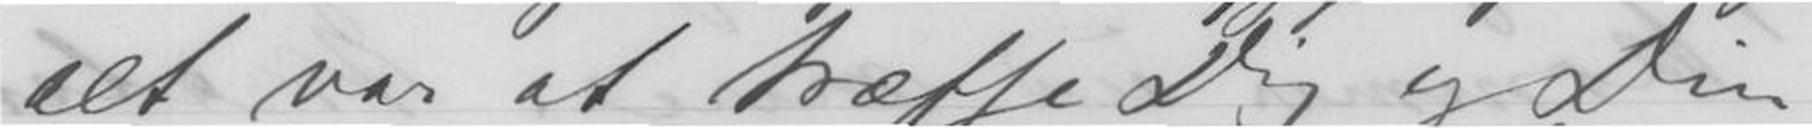alt var at træffe Dig og Din
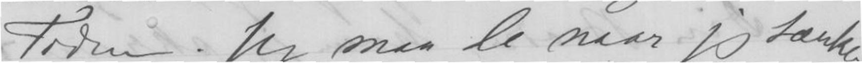Tiden. Jeg maa le naar jeg tænker
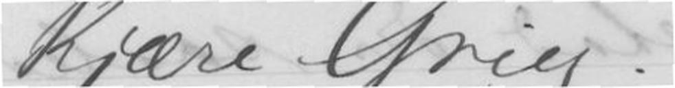Kjære Grieg!
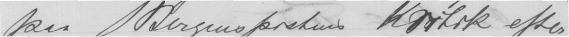paa Bergenspostens Kritik efter
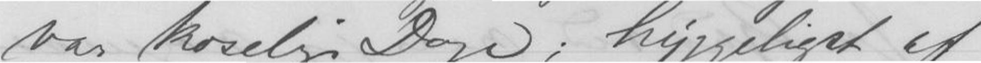var koselige Dage; hyggeligst af
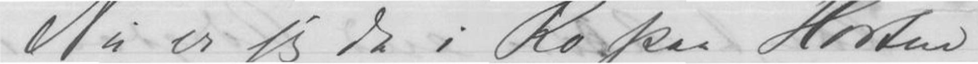Nu er jeg da i Ro paa Horten
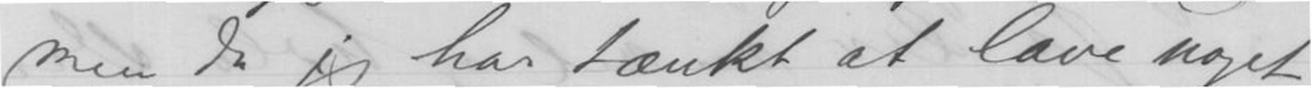men da jeg har tænkt at lave noget
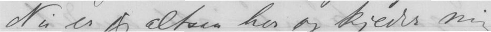Nu er jeg altsaa her og kjeder mig,
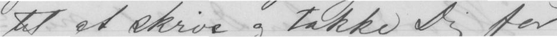til at skrive og takke Dig for
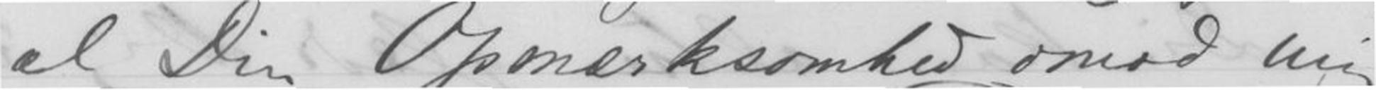al Din Opmærksomhed imod mig
